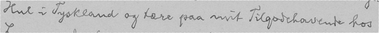Hul i Tyskland og tære paa mit Tilgodehavende hos
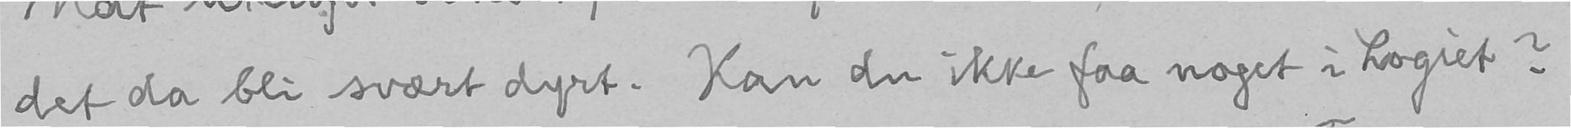det da bli svært dyrt. Kan du ikke faa noget i Logiet?
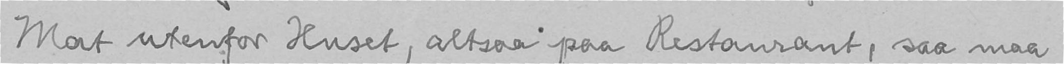Mat utenfor Huset, altsaa paa Restaurant, saa maa
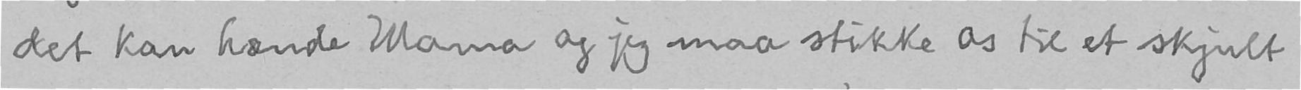det kan hænde Mama og jeg maa stikke os til et skjult
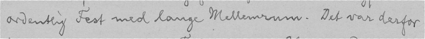ordentlig Fest med lange Mellemrum. Det var derfor
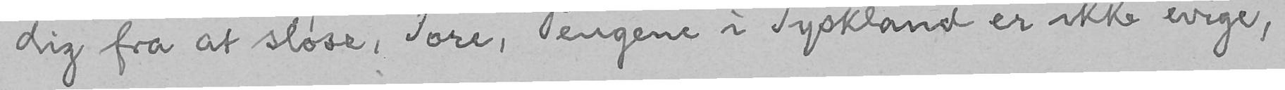dig fra at sløse, Tore, Pengene i Tyskland er ikke evige,
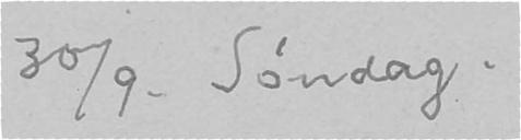30/9. Søndag.
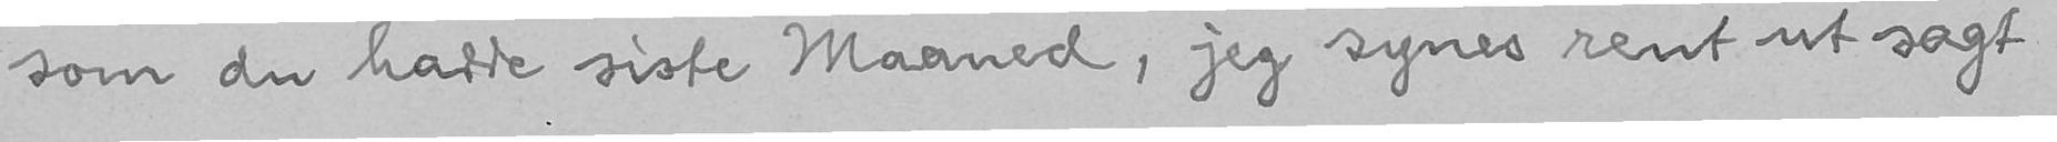som du hadde siste Maaned, jeg synes rent ut sagt
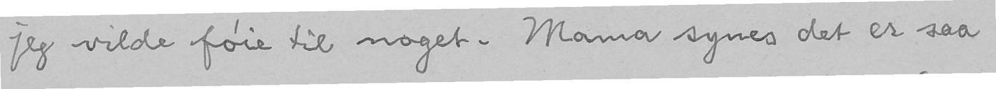jeg vilde føie til noget. Mama synes det er saa
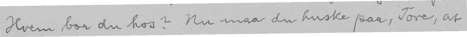Hvem bor du hos? Nu maa du huske paa, Tore, at
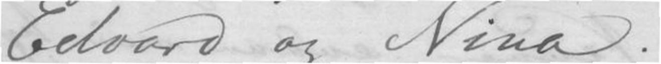Edvard og Nina.
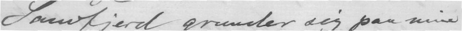Sandfjord grunder sig paa min
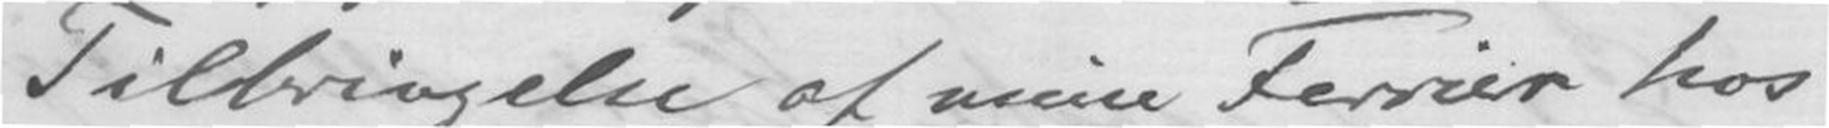Tilbringelse af mine Ferrier hos
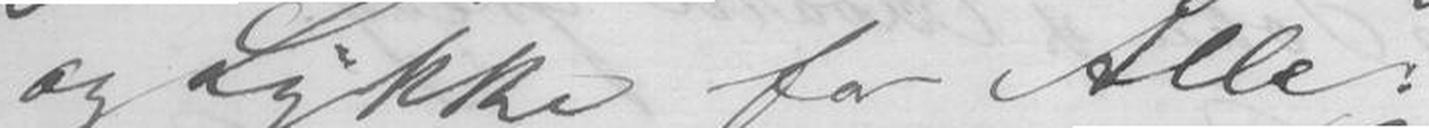og Lykke for Alle.
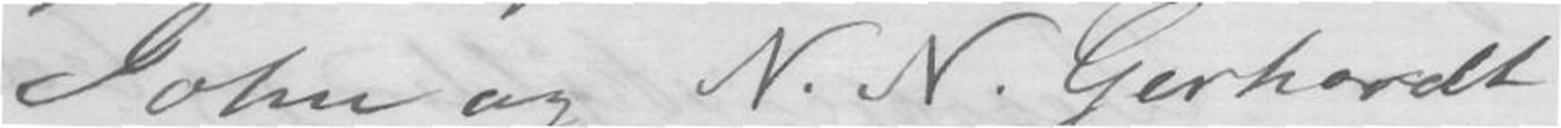John og N. N. Gerhordt
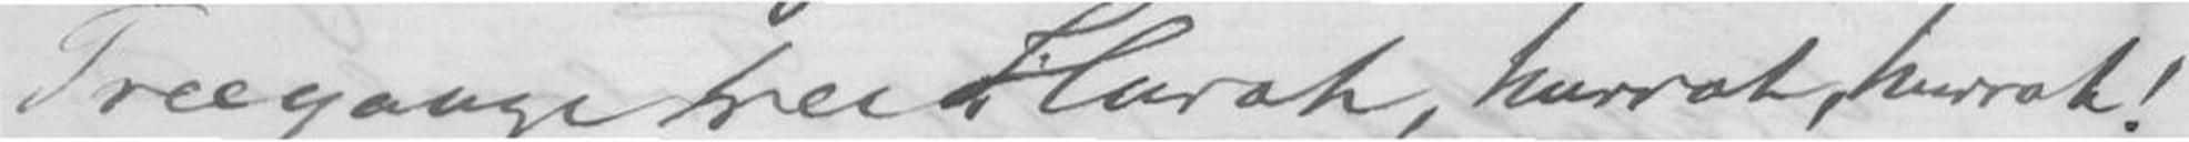Treegange tree Hurah, hurrah, hurrah!
